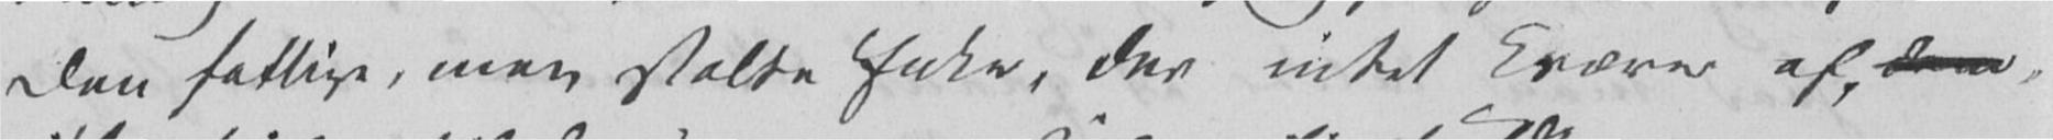Den fattige, men stolte Enke, der intet kræves af, dem,
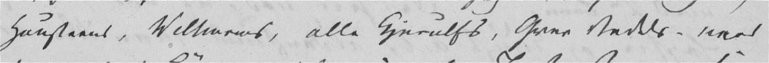Hansteens, Welhavens, alle Kjerulfs, Grev Vedels – naar
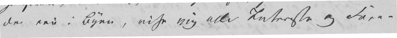de ere i Byen, vise mig alle Interesse og Fore-
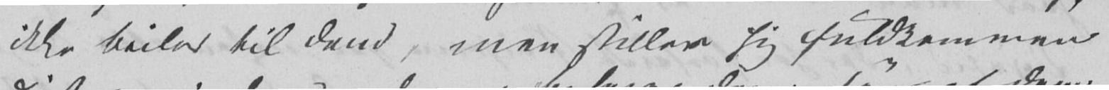ikke beiler til dem, men stiller sig fuldkommen
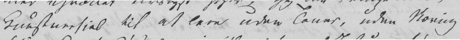Kunstnersjel til at leve uden Toner, uden Næring,
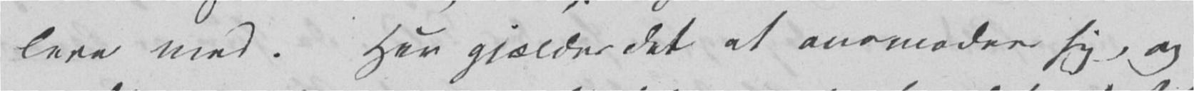leve med. Her gjælder det at accomodere sig, og
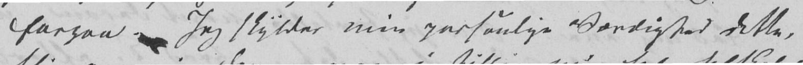forgaa. Jeg skylder min personlige Værdighed dette,
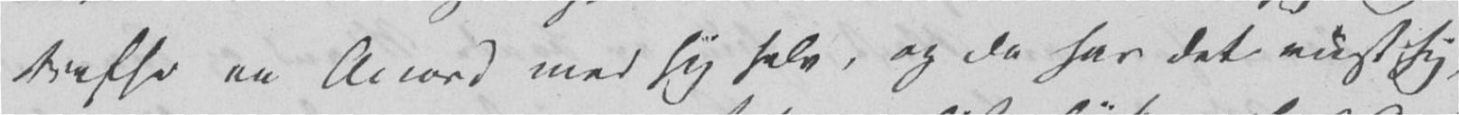treffe en Accord med sig selv, og da har det viist sig,
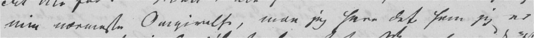min nærmeste Omgivelse, maa jeg have det som jeg er
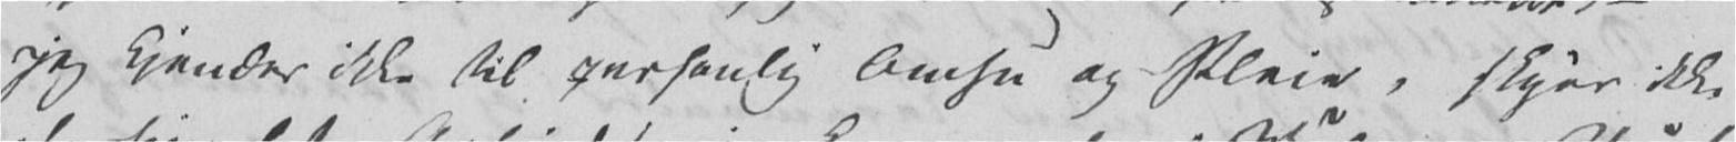jeg kjender ikke til personlig Omhu og Pleie, skyer ikke
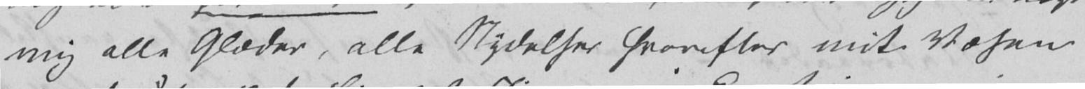mig alle Glæder, alle Nydelser hvorefter mit Væsen
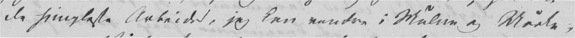de simpleste Arbeider, jeg kan vandre i Mulm og Mørke,
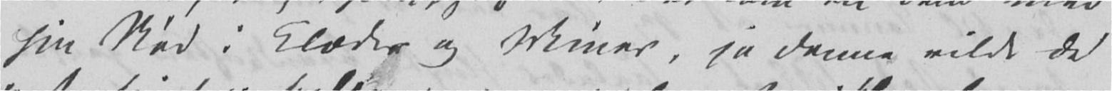sin Nød i Klæder og Miner, ja denne vilde de
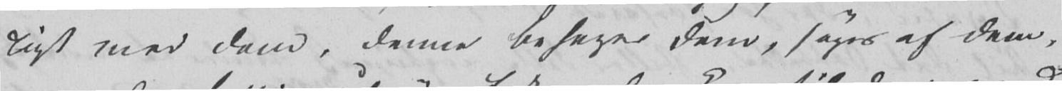ligt med dem, denne behager dem, søges af dem,
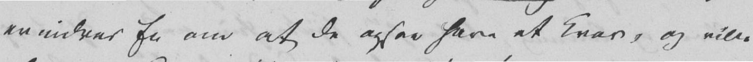erindrer En om at de ogsaa have et Krav, og ville
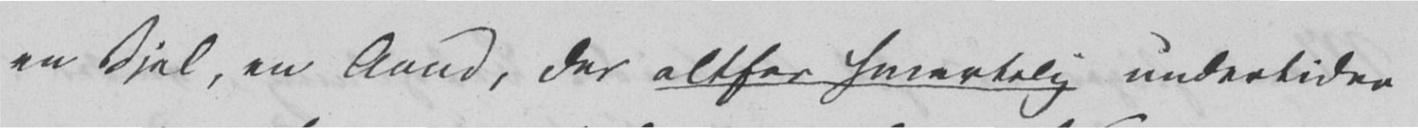en Sjel, en Aand, der altfor smertelig undertiden
